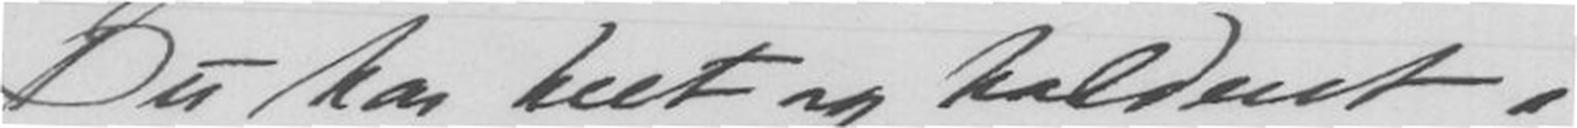Du har helt og holdent å
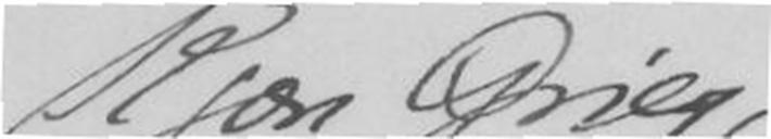Kjære Grieg,
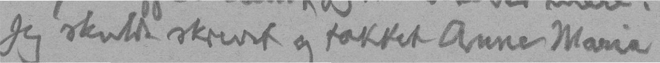Jeg skulde skrevet og takket Anne Marie
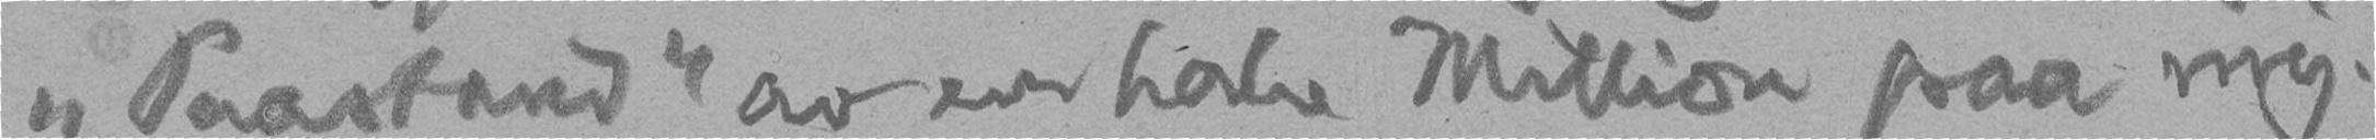"Paastand" av en halv Million paa mig.
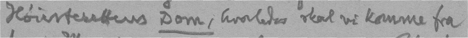Høiesterettens Dom, hvorledes skal vi komme fra
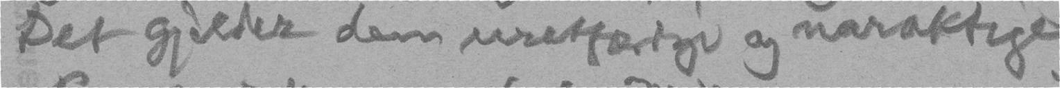Det gjelder den uretfærdige og naraktige
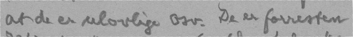at de er ulovlige osv. De er forresten
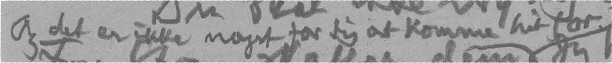Og det er ikke noget for dig at komme hit for
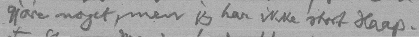gjøre noget, men jeg har ikke stort Haap.
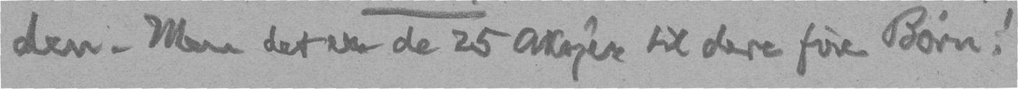den. Men det er de 25 Aksjer til dere frie Børn!
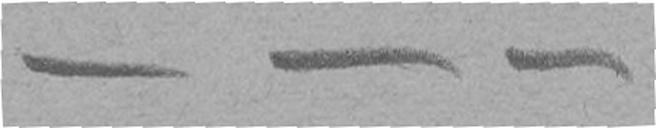- - -
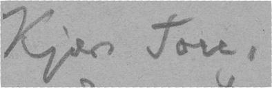Kjære Tore,
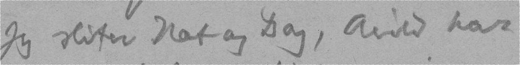Jeg sliter Nat og Dag, Arild har
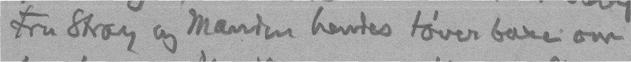Fru Stray og Manden hendes tøver bare om
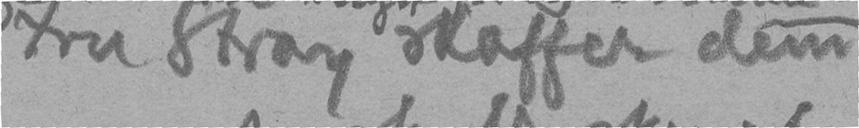Fru Stray skaffer dem
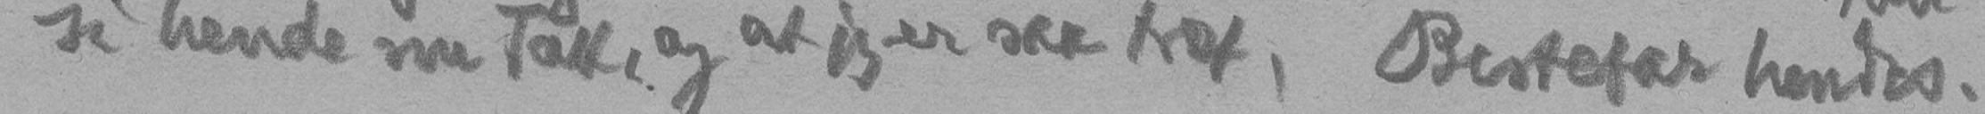si hende nu Tak, og at jeg er saa træt. Bestefar hendes.
